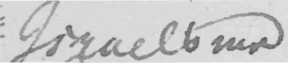Giraelbma
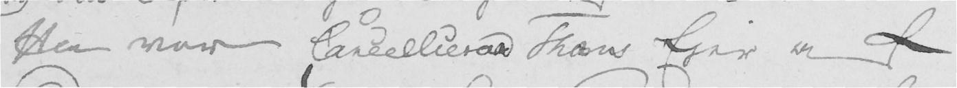Her var Cancellieraad Hans Ejer af
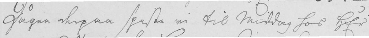Dagen derpaa spiste vi til Middag hos Hr
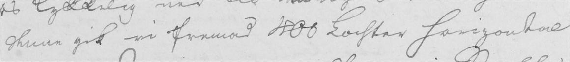denne gik vi fremad 400 Lachter horizontal
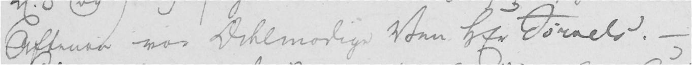Aftenen vor Ædelmodige Ven Hr Tøraels. -
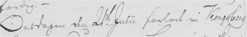Onsdagen den 21te Julii forlode vi Kongsberg
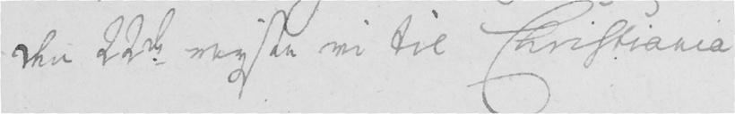den 22de reyste vi til Christiania
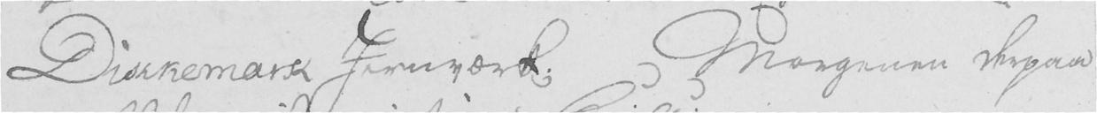Dikkemark Jernværk; Morgenen derpaa
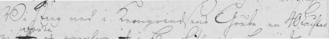Vi steeg ned i Kronprindsens Grube en 40 Lachter
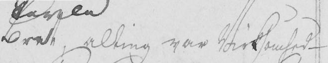Bret, alting var Virkomhed -
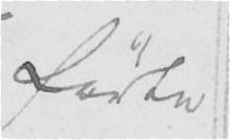førte
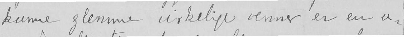kunne glemme virkelige venner er en u-
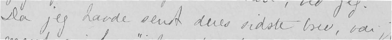Da jeg havde sendt deres sidste brev, var jeg
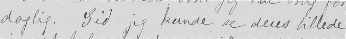daglig. Gid jeg kunde se deres billede,
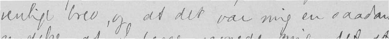venlige brev, og at det var mig en saadan
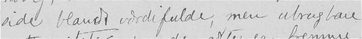side blandt værdifulde, men ubrugbare
